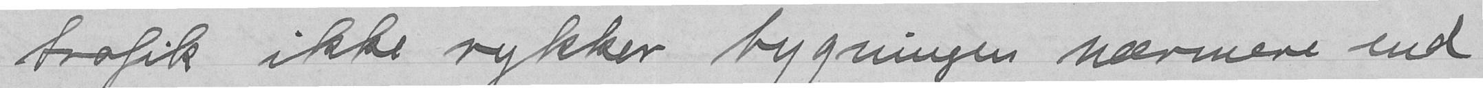trafik ikke rykker bygningen nærmere end
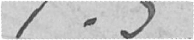P.S
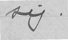sig
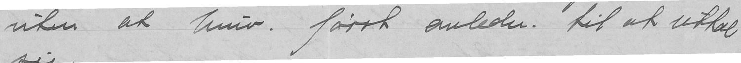uten at Univ. først anledn. til at uttale
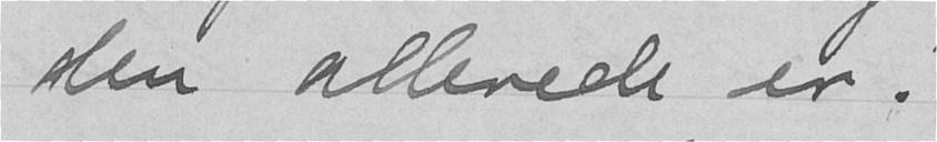den allerede er.
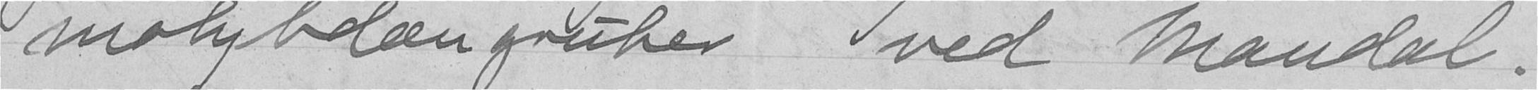molybdængruber ved Mandal.
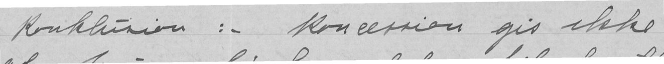Konklusion: - Koncession gis ikke
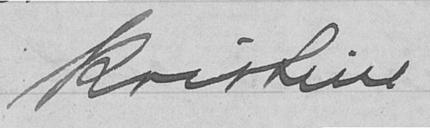Kristine
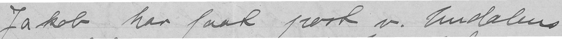Jakob har faat post v. Undalens
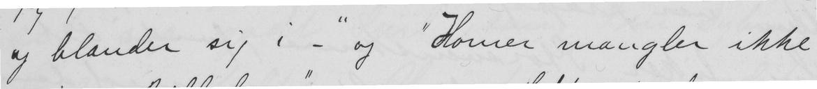og blander sig i -" og "Homer mangler ikke
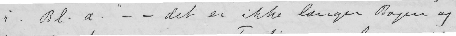i. Bl. a. "- - det er ikke længer Bogen og
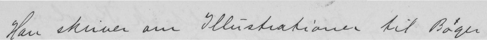Han skriver om Illustrationer til Bøger
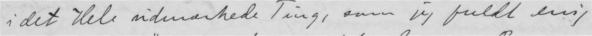i det Hele udmærkede Ting, som jeg fuldt enig
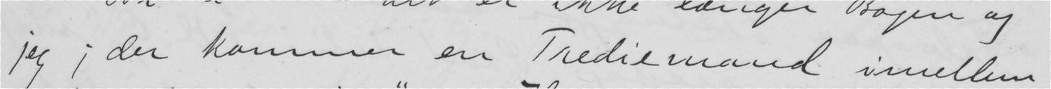jeg; der kommer en Trediemand imellem
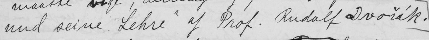und seine Lehre" af Prof. Rudolf Dvoiák.
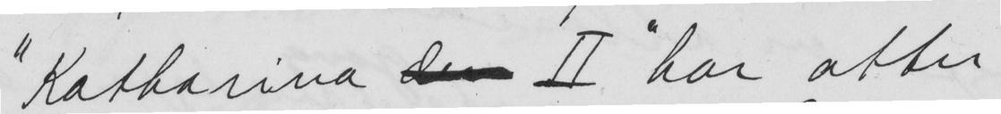"Katharina  2" har atter
